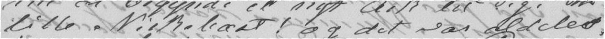lille Niskebarn! og det var aldeles
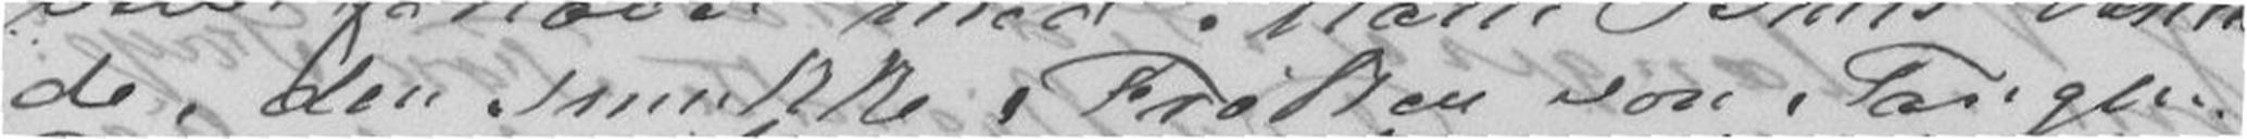de, den smukke Frøken von Tangen.
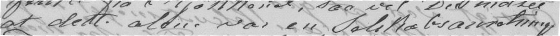at dette alene var en Sælskabsanretning.
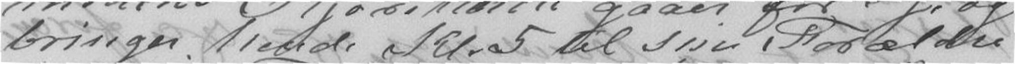bringer hende Kl. 5 til sine Forældre
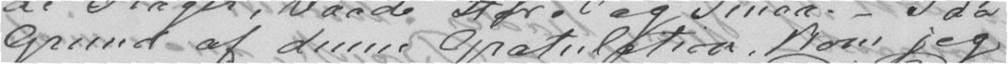Grund af denne Gratulation kom jeg
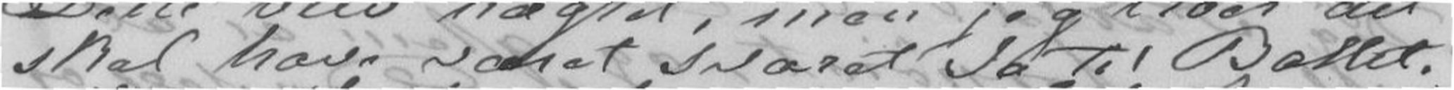skal have været svaret Ja til Ballet.
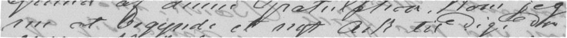nu at begynde et nyt Ark til Dig. Du
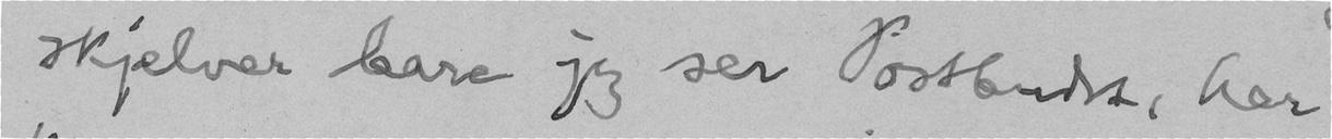skjelver bare jeg ser Postbudet, her
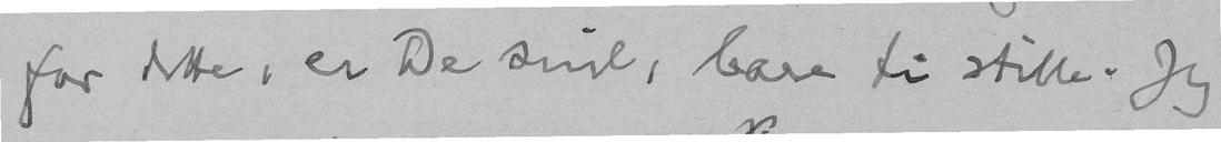for dette, er De snil, bare ti stille. Jeg
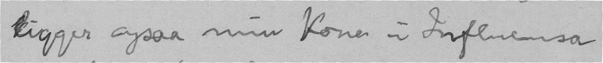ligger ogsaa min Kone i Influensa
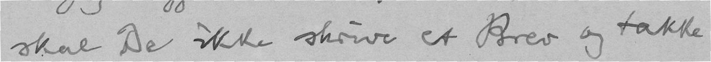skal De ikke skrive et Brev og takke
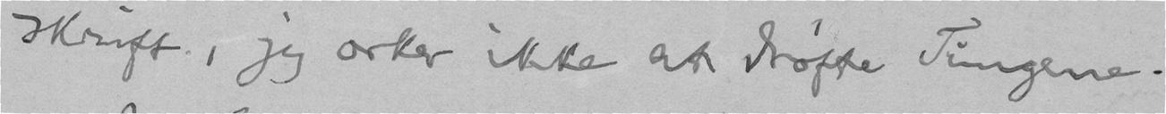skrift, jeg orker ikke at drøfte Tingene.
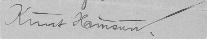Knut Hamsun.
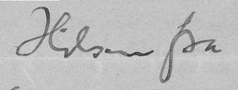Hilsen fra
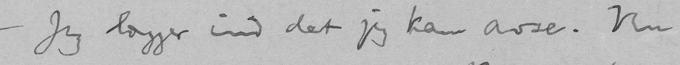- Jeg lægger ind det jeg kan avse. Nu
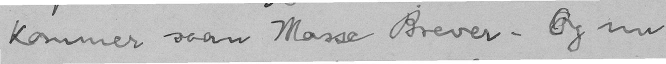kommer saan Masse Brever. Og nu
